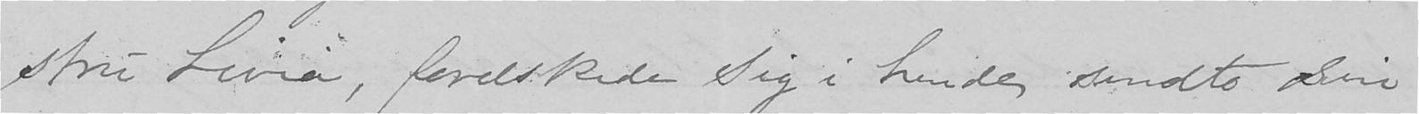stru Livia, forelskede sig i hende, sendte sin
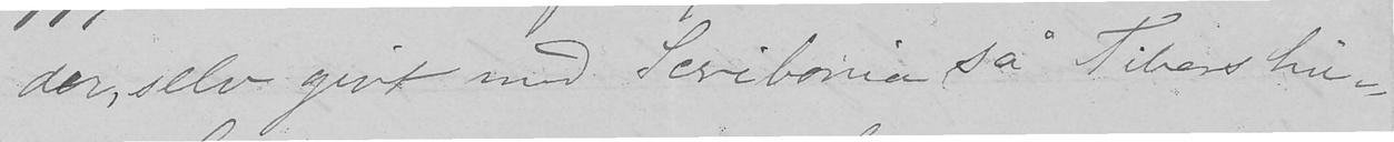der, selv givt med Scribonia så Tibers hu-
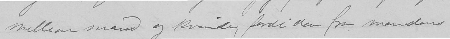mellem mand og kvinde, fordi den fra mandens
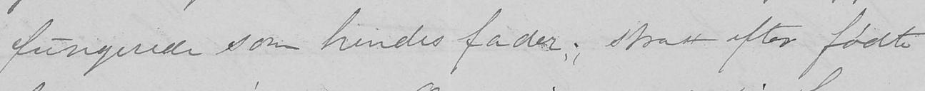fungerede som hendes fader; strax efter fødte
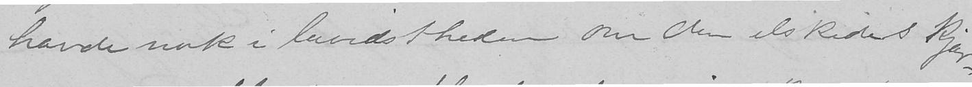havde nok i bevidstheden om den elskedes kjær-
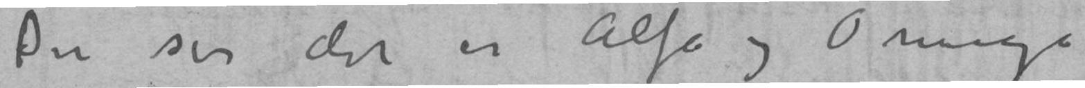Du ser det er Alfa og Omega
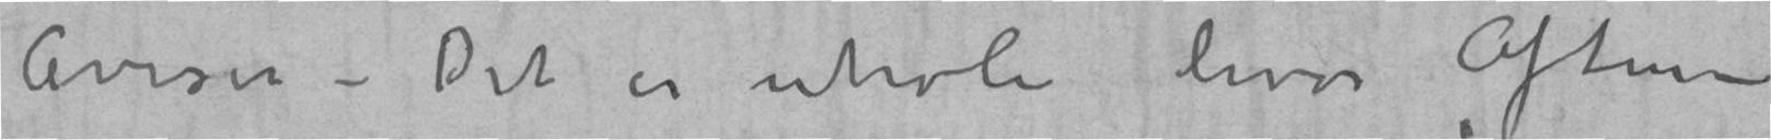Avisen – Det er utroli hvor Aften
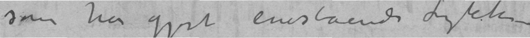som har gjort enestaaende Lykke –
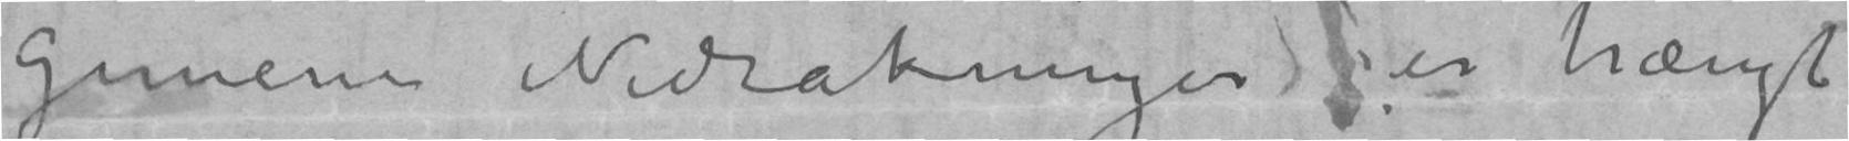gemene Nedrakninger er trængt
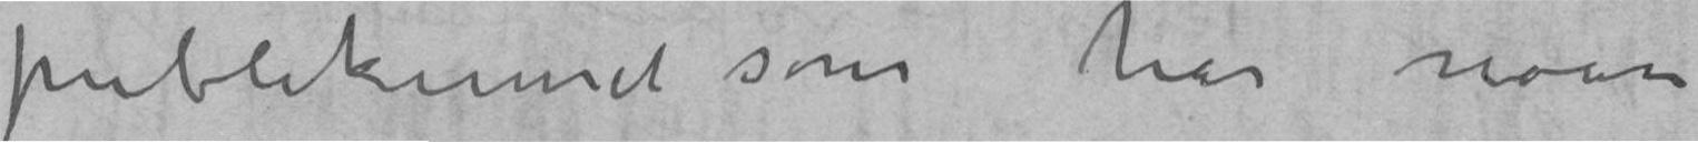publikumet som har noen
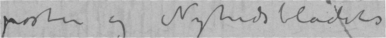posten og Nyhedsbladets
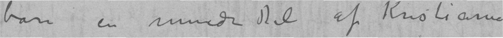bare en mindre Del af Kristiania
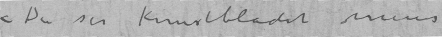–	Du ser Kunstbladet mener
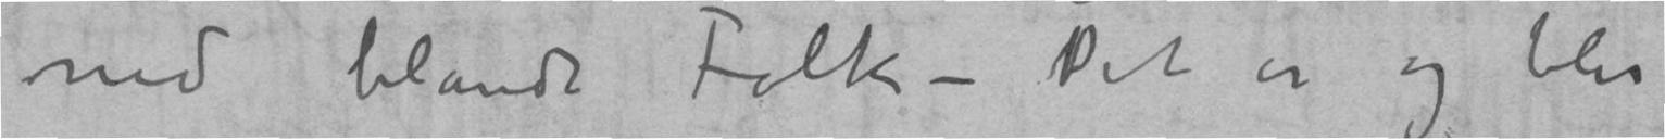ind blandt Folk – Det er og blir
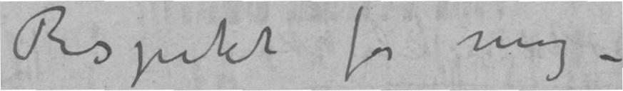Respekt for mig –
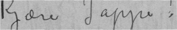Kjære Jappe!
